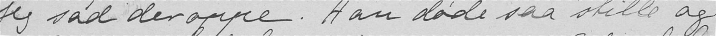jeg sad der oppe. Han døde saa stille og
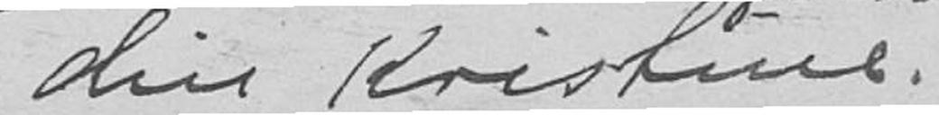din Kristine.
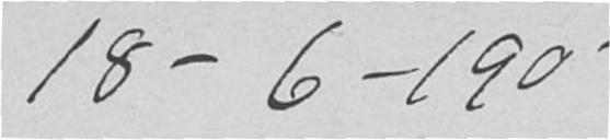18-6-1903
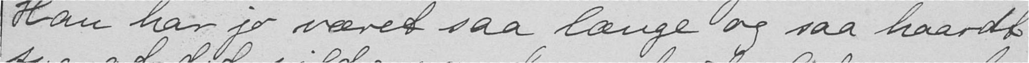Han har jo været saa længe og saa haardt
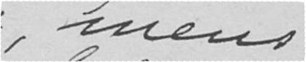mens
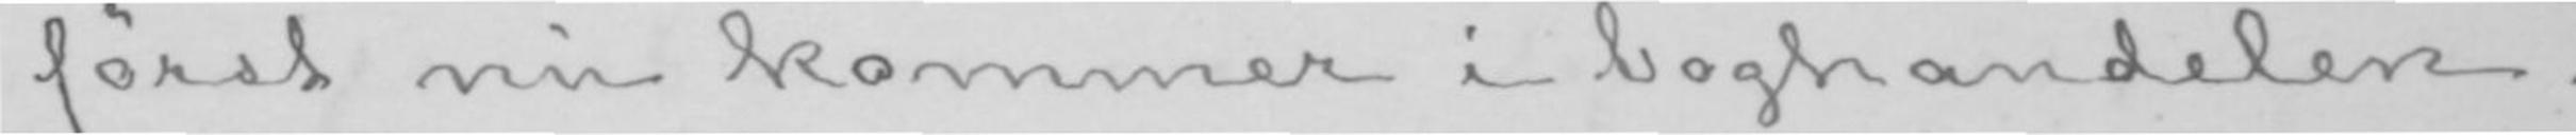først nu kommer i boghandelen.
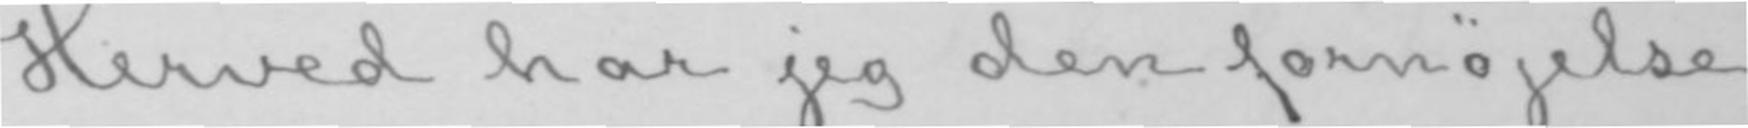Herved har jeg den fornøjelse
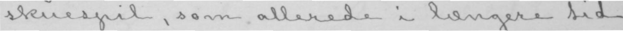skuespil, som allerede i længere tid
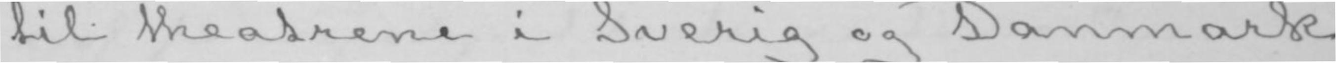til theatrene i Sverig og Danmark
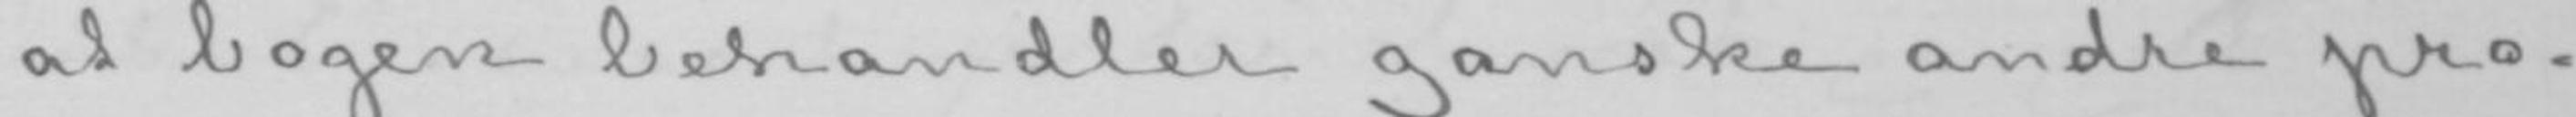at bogen behandler ganske andre pro-
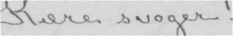Kære svoger!
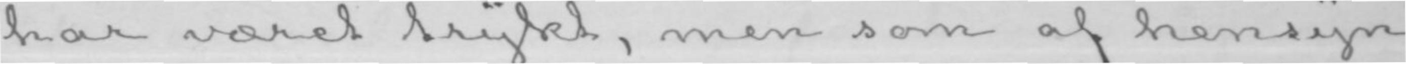har været trykt, men som af hensyn
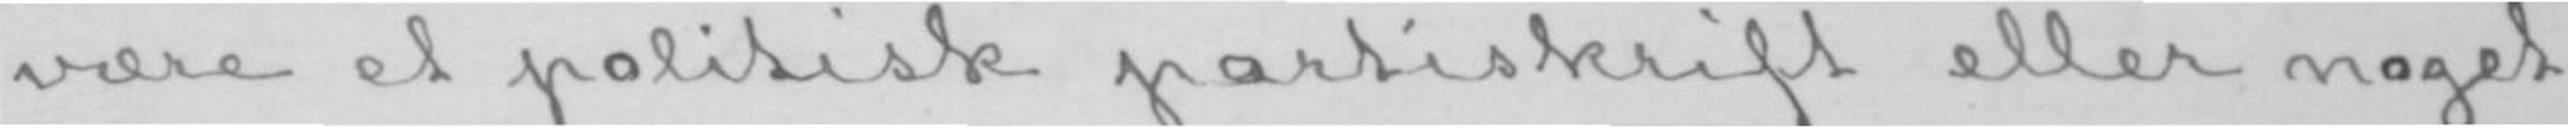være et politisk partiskrift eller noget
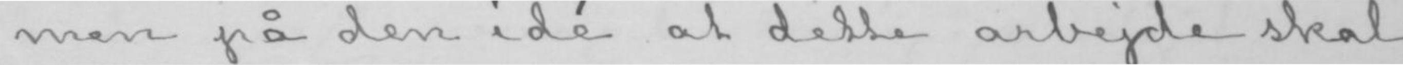men på den idè at dette arbejde skal
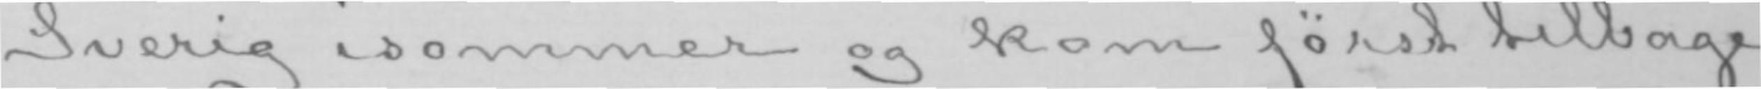Sverig isommer og kom først tilbage
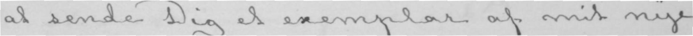at sende Dig et exemplar af mit nye
